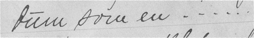dum som en ......
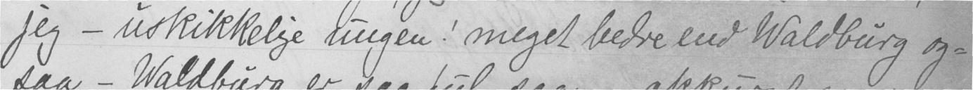jeg - uskikkelige ungen! meget bedre end Waldburg og-
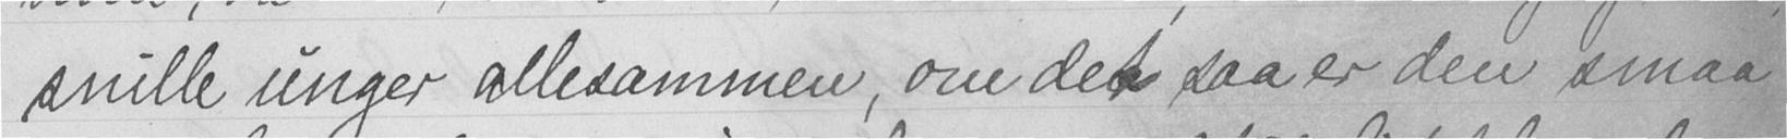snille unger allesammen, om det saa er den smaa
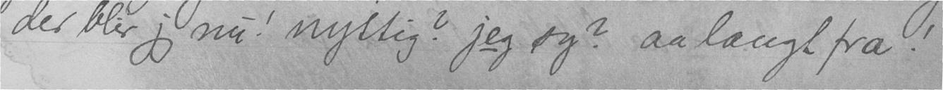der blir jeg nu! nyttig? jeg sy? aa langt fra!
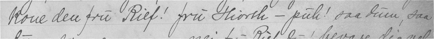kone den fru Rief! fru Hiorth - puh! saa dum, saa
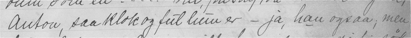Anton, saa klok og ful hun er - ja, han ogsaa; men
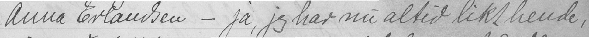Anna Erlandsen - ja, jeg har nu altid likt hende,
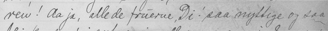ren! aa ja, alle de fruerne, Di! saa nyttige og saa
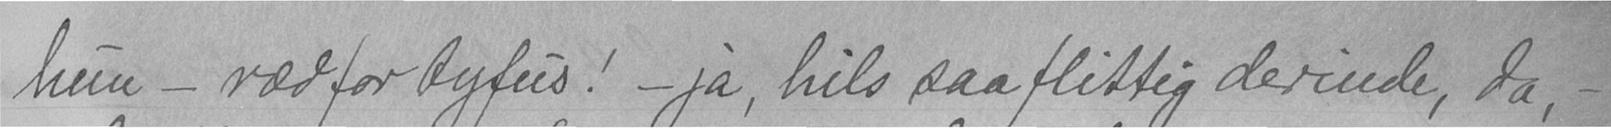hun - ræd for tyfus! - ja, hils, saa flittig derinde, da, -
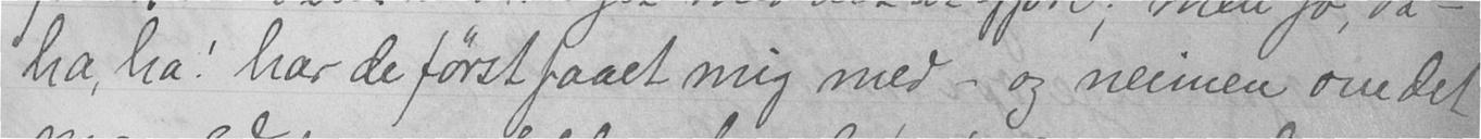ha, ha! har de først faaet mig med - og neimen om det
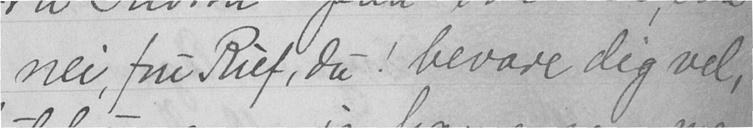nei, fru Rief, du! bevare dig vel,
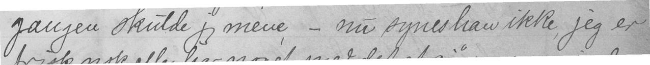gangen skulde jeg mene, - nu synes han ikke, jeg er
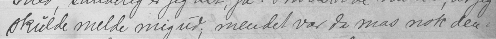skulde melde mig ud; mendet var da mas nok den
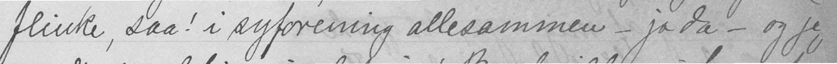flinke, saa! i syforening allesammen - ja da - og jeg
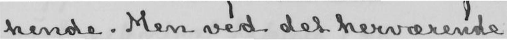hende. Men ved det herværende
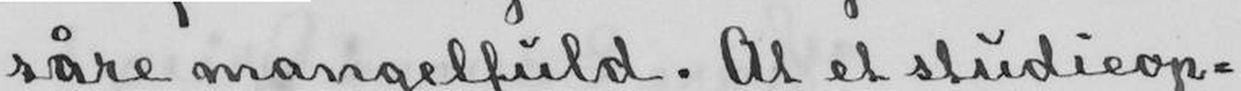såre mangelfuld. At et studieop-
